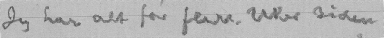Jeg har alt for flere Uker siden
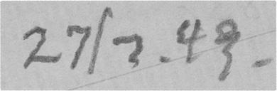27/7. 49.
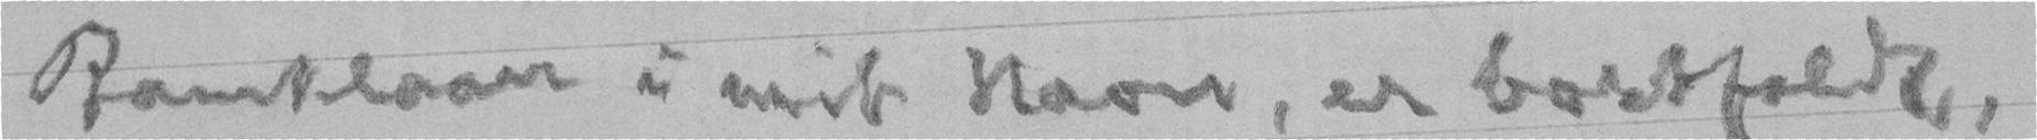Banklaan i mit Navn, er bortfaldt,
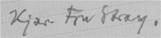Kjære Fru Stray,
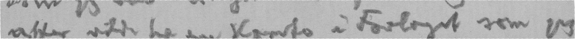atter vilde ha en konto i Forlaget som jeg
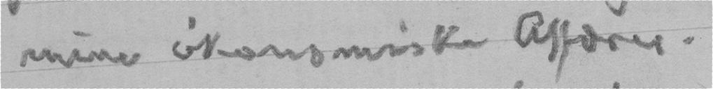mine økonomiske Affærer.
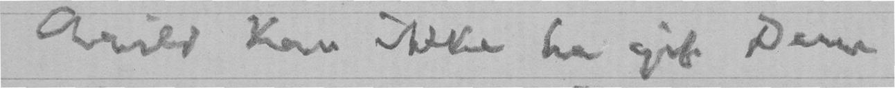Arild kan ikke ha git Dem
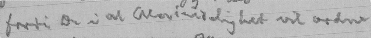fordi De i al Almindelighet vil ordne
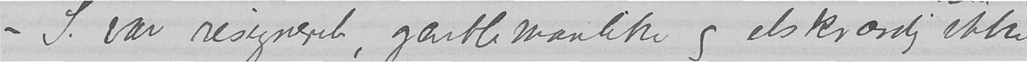S. var resigneret, gentlemanlike og elskværdig som ikke
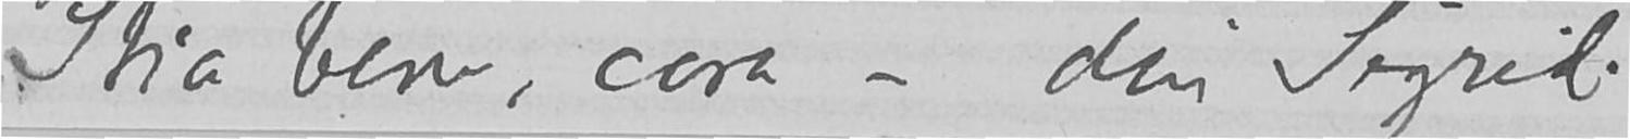Stia bene, cora - din Sigrid.
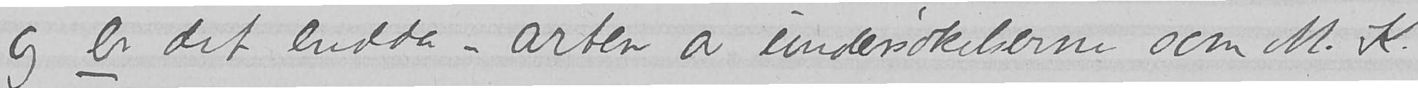og er det endda – arten av undersøkelserne som M.K.
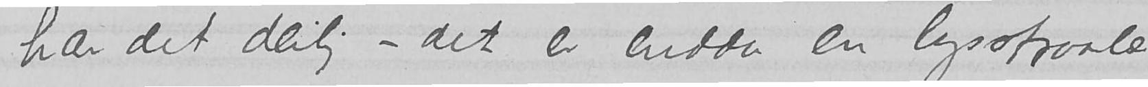og har det deilig – det er endda en lysstraale.
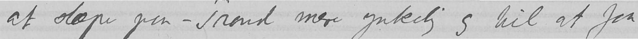at slæpe paa –Trond mere ynkelig og til at faa
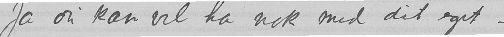Ja du kan vel ha nok med dit eget –
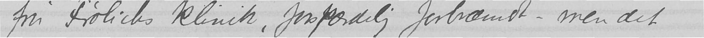fru Frølichs klinik, forfærdelig forbrændt – men det
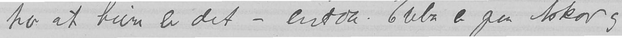tror at hun er det –endda. Ebba er paa Askov
